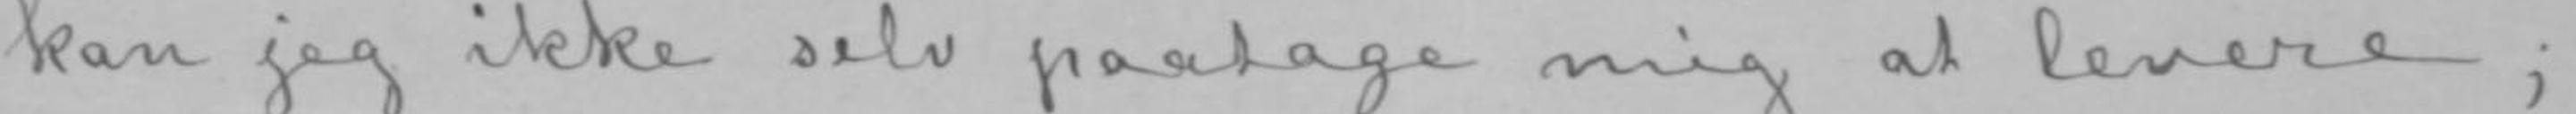kan jeg ikke selv paatage mig at levere;
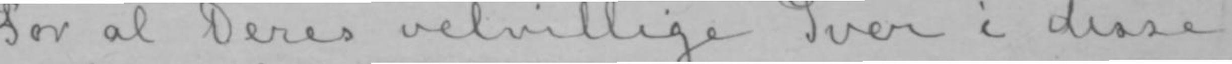For al Deres velvillige Iver i disse
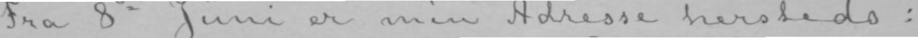Fra 8de Juni er min Adresse hersteds:
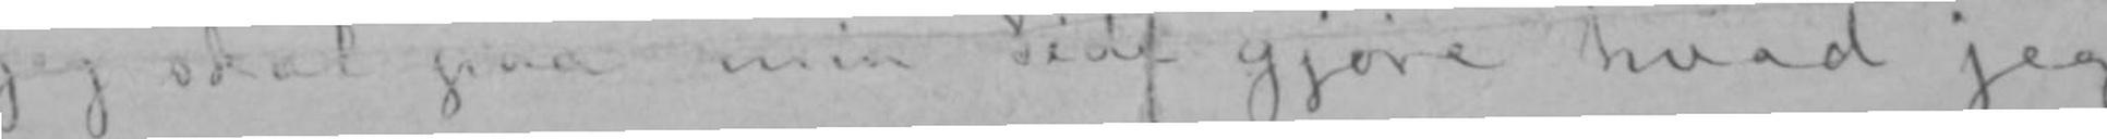Jeg skal paa min Side gjøre hvad jeg
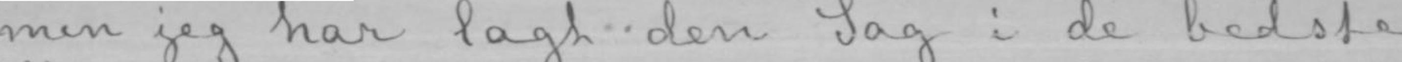men jeg har lagt den Sag i de bedste
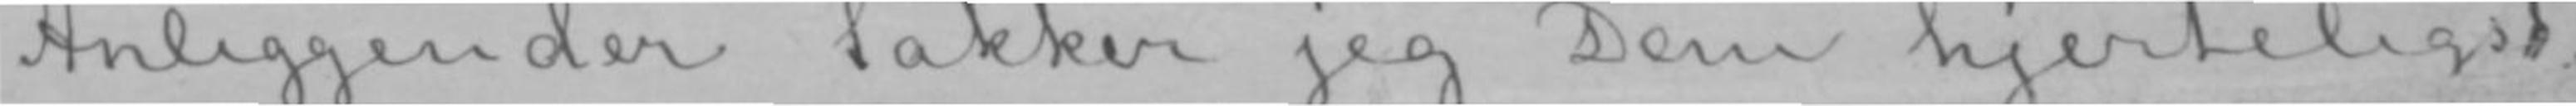Anliggender takker jeg Dem hjerteligst.
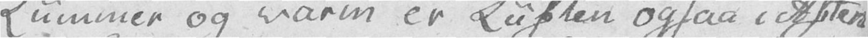Lummer og varm er Luften ogsaa i Aften
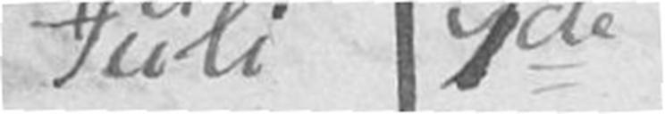Juli 7de
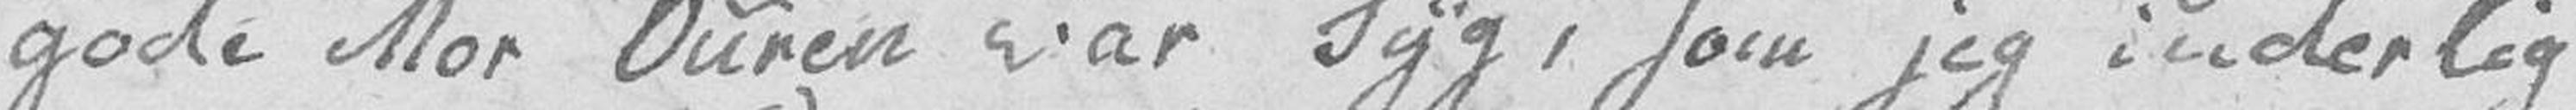gode Mor Ouren var Syg, som jeg inderlig
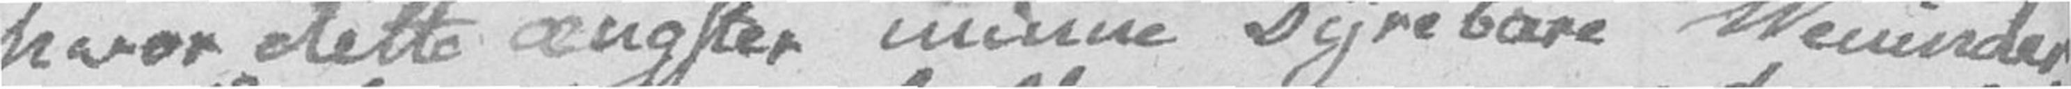hvor dette ængster minne Dyrebare Veninder
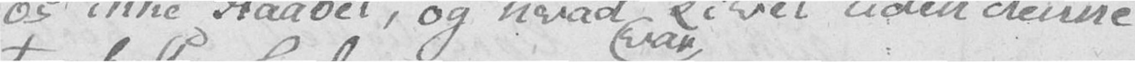os ikke Haabet, og hvad var Livet uden denne
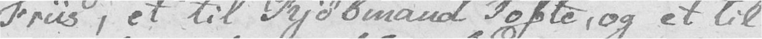Friis, et til Kjøbmand Tofte, og et til
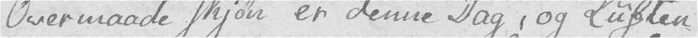Overmaade skjøn er denne Dag, og Luften
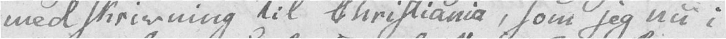med skrivning til Christiania, som jeg nu i
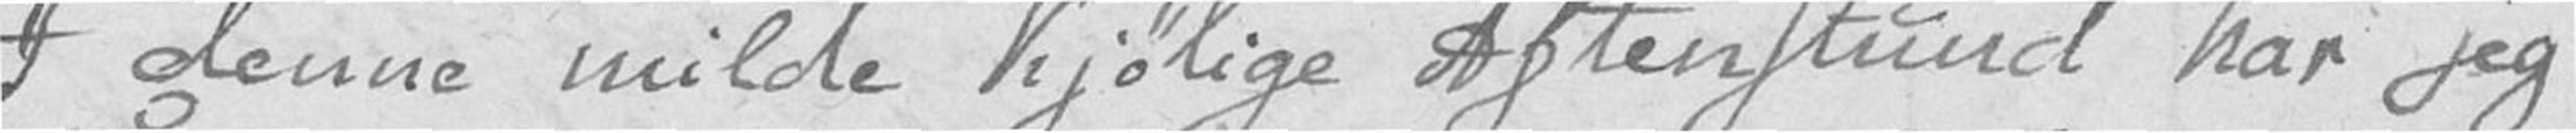I denne milde kjølige Aftenstund har jeg
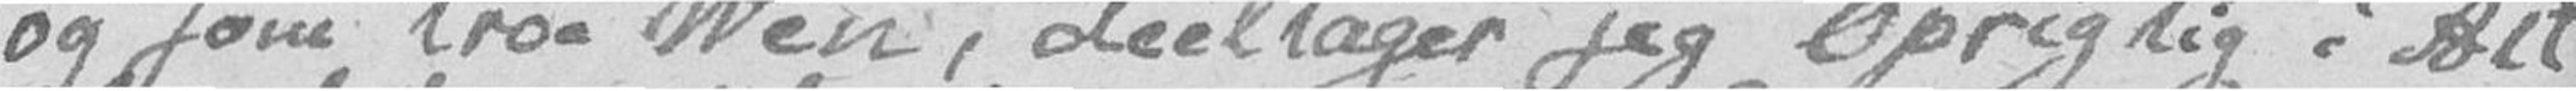og som troe Ven, deeltager jeg Oprigtig i Alt
